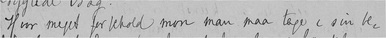Hvor meget forbehold mon man maa tage i sin be-
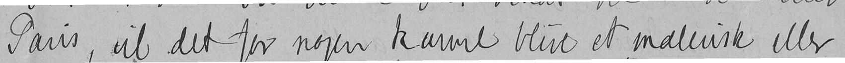Paris, vil det for nogen kunne blive et malerisk eller
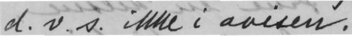d.v.s. ikke i avisen.
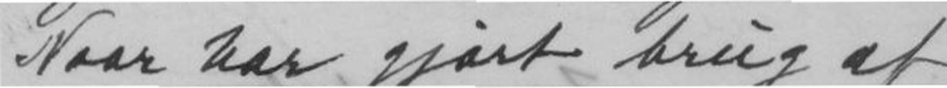Naar har gjort brug af
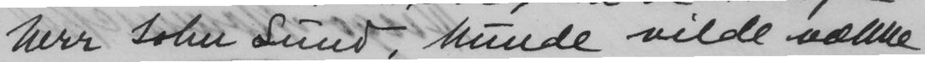herr John Lund, kunde vilde vække
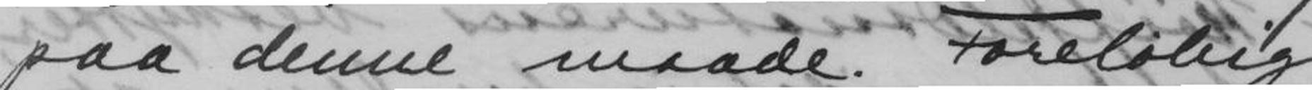paa denne maade. Foreløbig
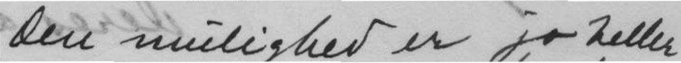Den mulighed er jo heller
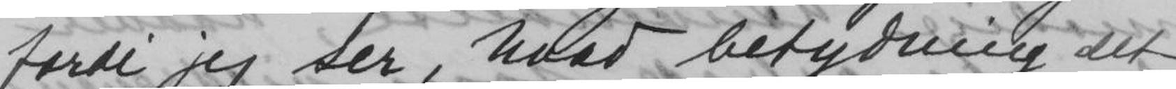fordi jeg ser, hvad betydning det
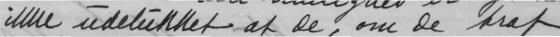ikke udelukket at De, om De traf
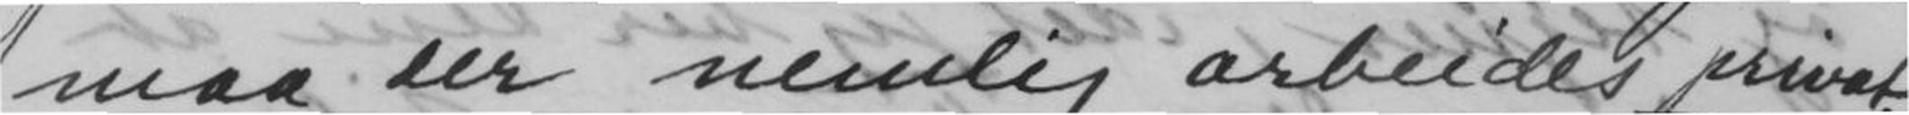maa der nemlig arbeides privat,
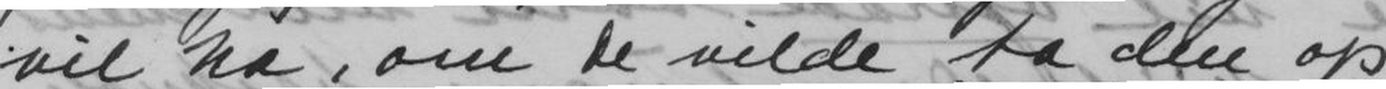vil ha, om De vilde ta den op
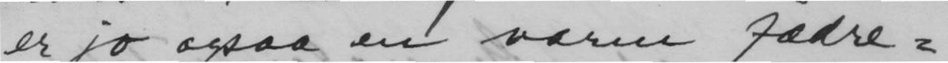er jo ogsaa en varm fædre-
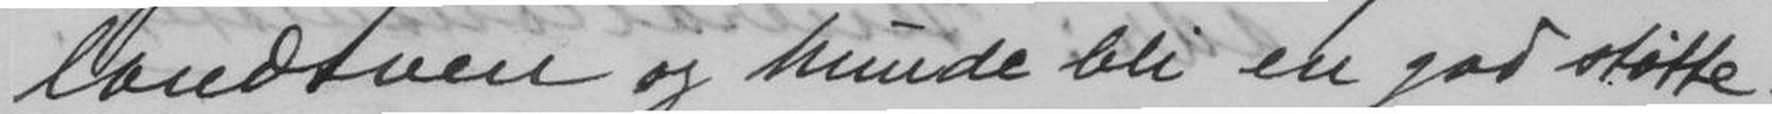landsven og kunde bli en god støtte.
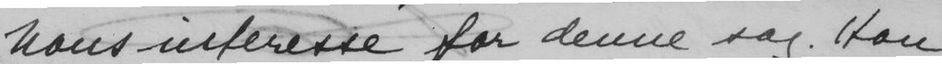hans interesse for denne sag. Han
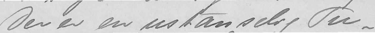Der er en ustanselig Tu-
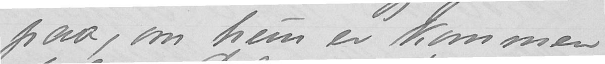paa, om hun er kommen
